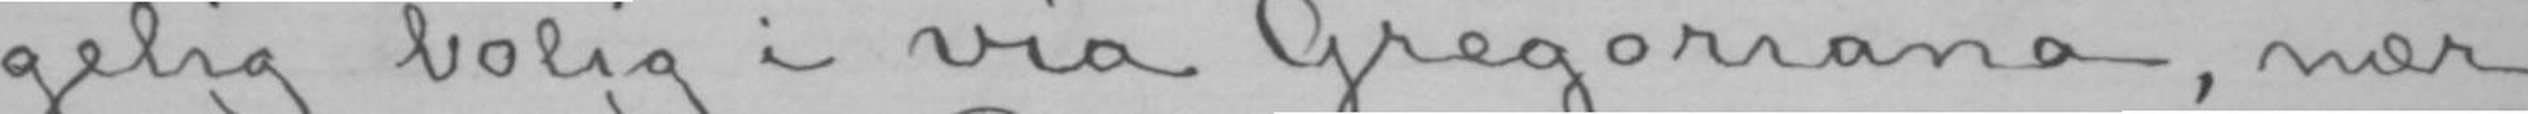gelig bolig i via Gregoriana, nær
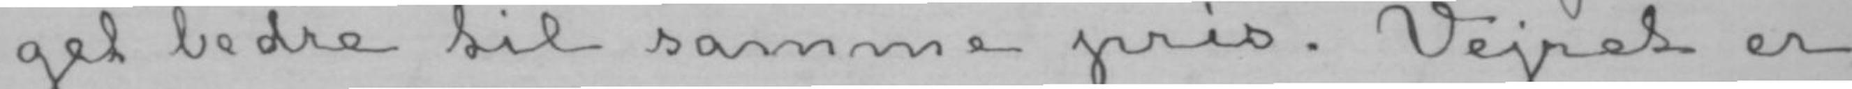get bedre til samme pris. Vejret er
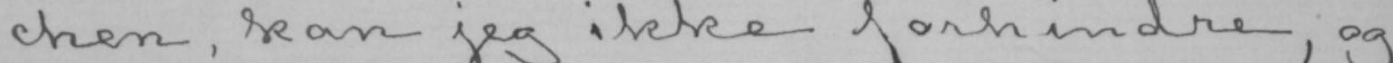chen, kan jeg ikke forhindre, og
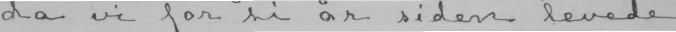da vi for ti år siden levede
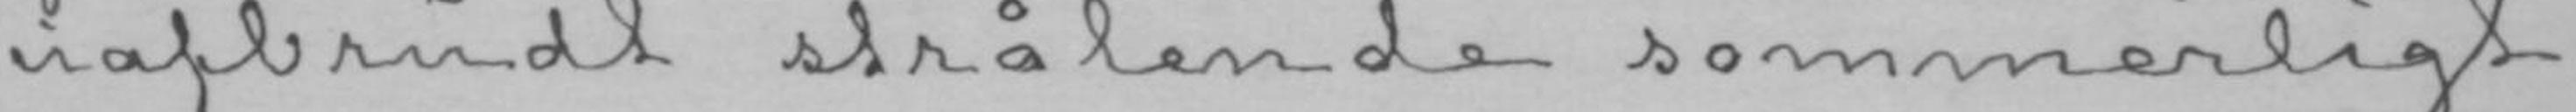uafbrudt strålende sommerligt,
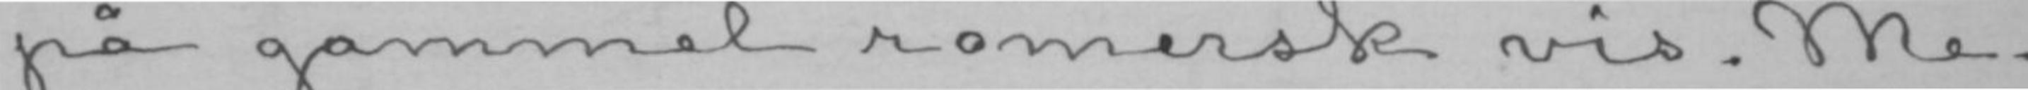på gammel romersk vis. Me-
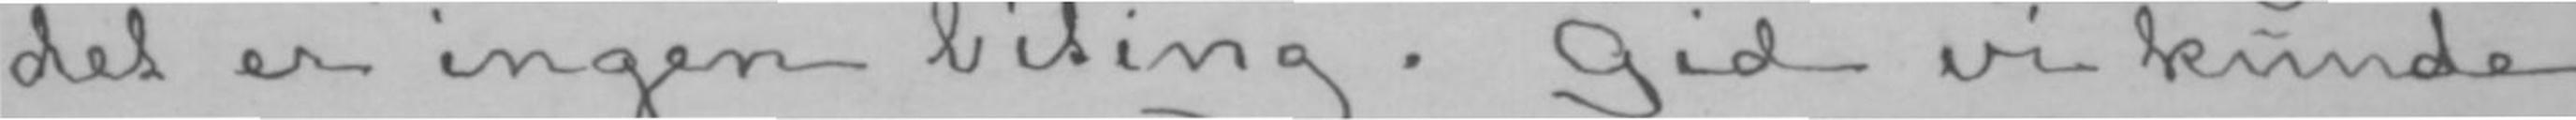det er ingen biting. Gid vi kunde
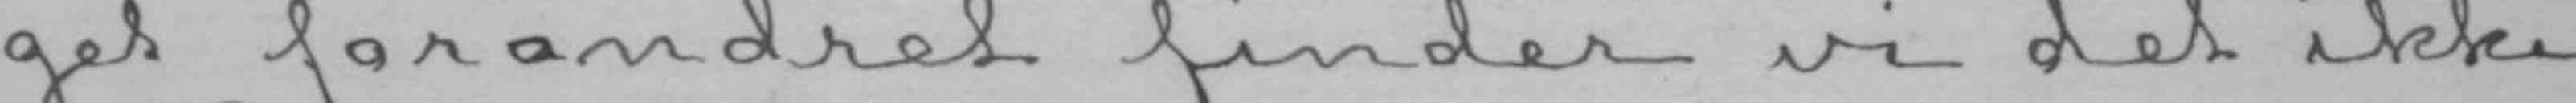get forandret finder vi det ikke
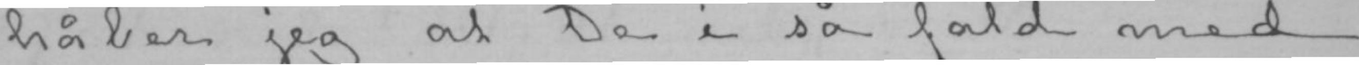håber jeg at De i så fald med
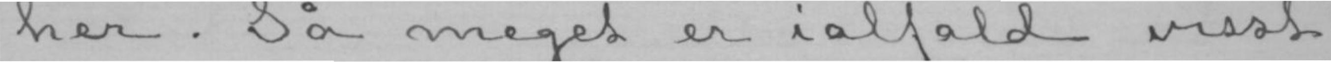her. Så meget er ialfald visst
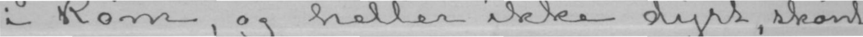i Rom, og heller ikke dyrt, skønt
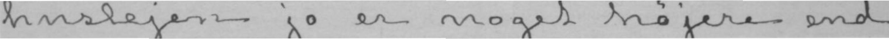huslejen jo er noget højere end
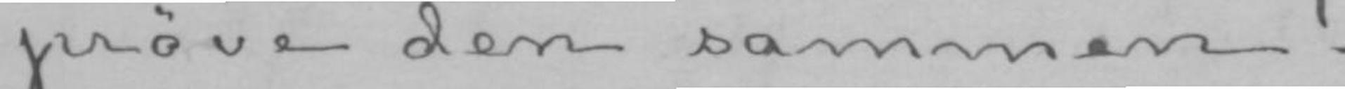prøve den sammen!
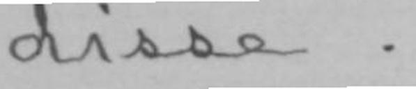disse.
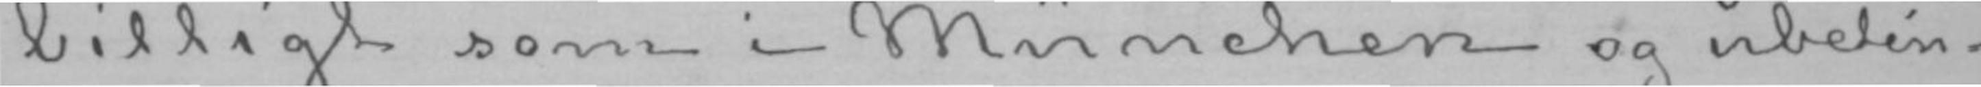billigt som i München og ubetin-
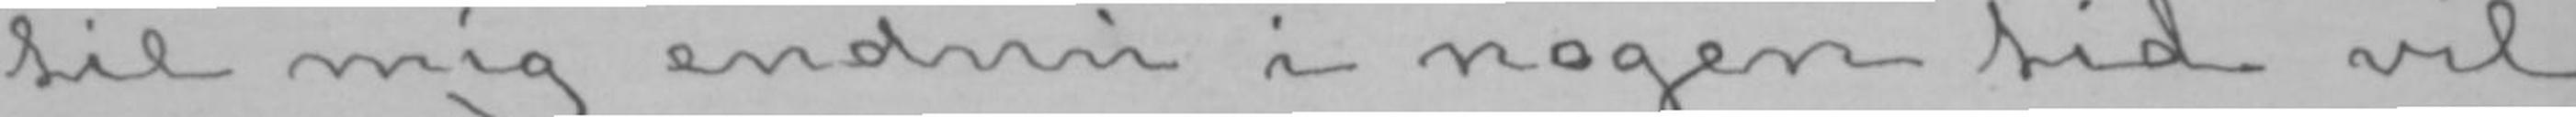til mig endnu i nogen tid vil
